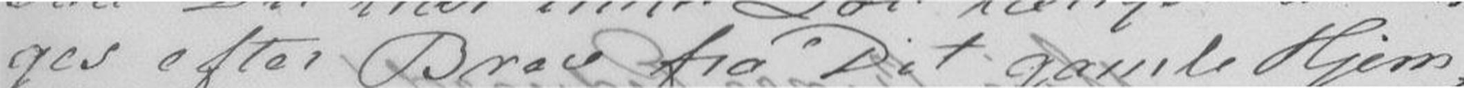ges efter Brev fra Dit gamle Hjem,
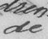de
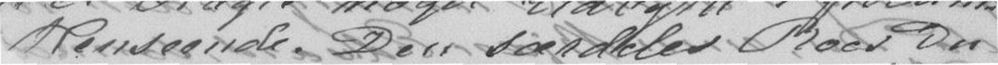Henseende. Den særdeles Roes Du
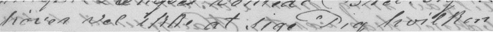høver vel ikke at sige Dig hvilken
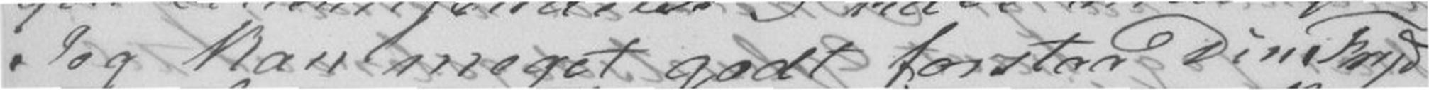Jeg kan meget godt forstaa Din Fryd
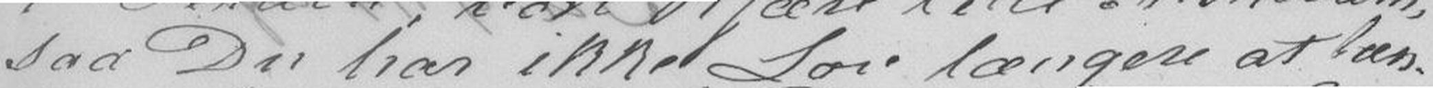saa Du har ikke Lov længere at læn-
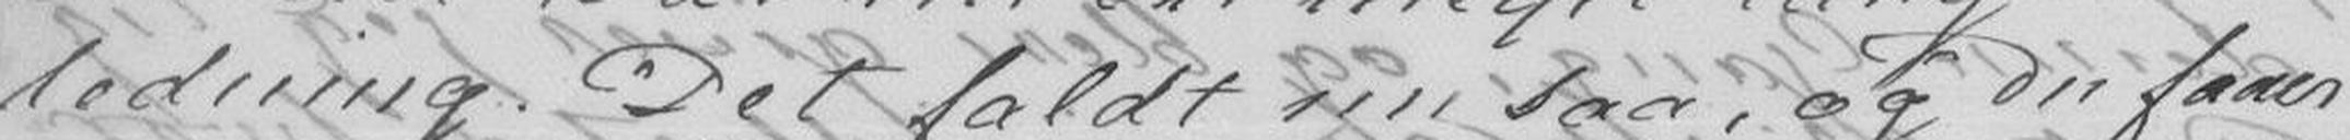ledning. Det faldt nu saa, og Du faaer
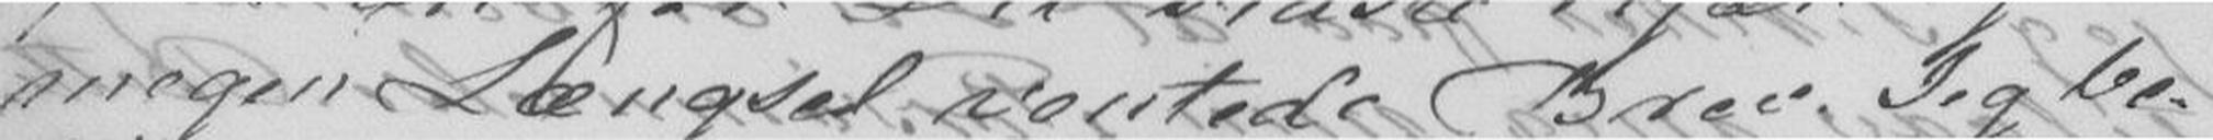megen Længsel ventede Brev. Jeg be-
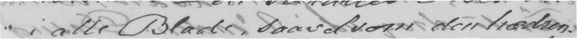i alle Blade saavelsom den hædren-
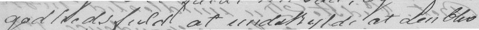godhedsfuld at undskylde at den blev
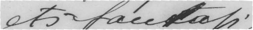ets fantasi
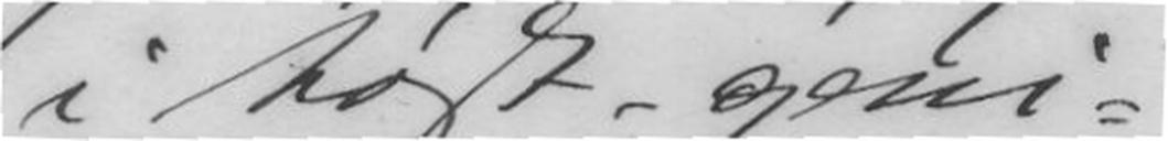i høst-geni-
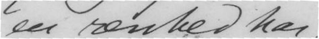en renhed har,
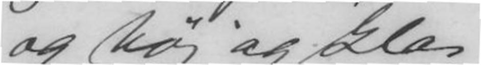og høj og klar
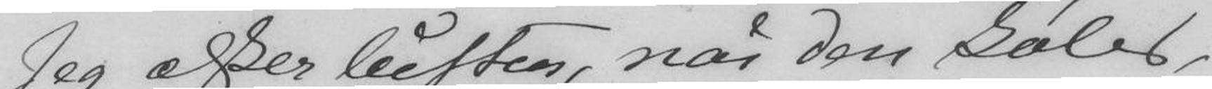Jeg ælsker luften, når den køles,
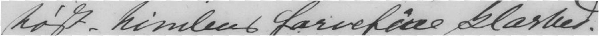høst-himlens farvefine klarhed.
